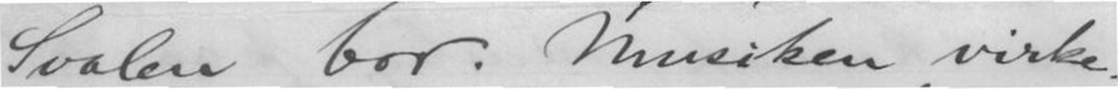Svalen bor. Musiken virke-
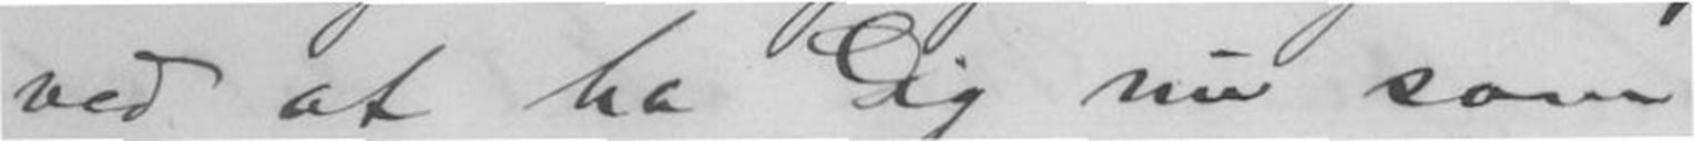ved at ha Dig nu som
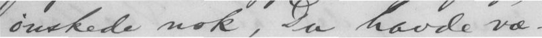ønskede nok, Du havde væ-
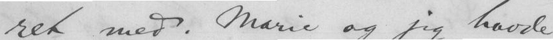ret med. Marie og jeg havde
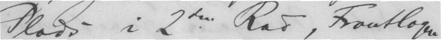Plads i 2den Rad, Frontlogen,
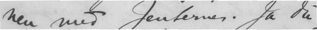nen med Jenterne. Ja Du
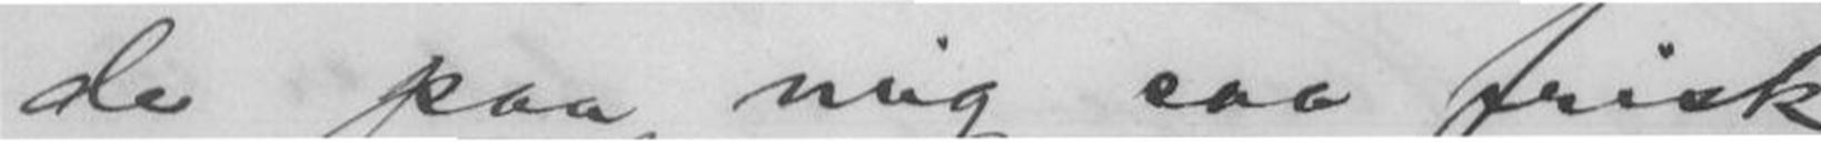de paa mig saa frisk
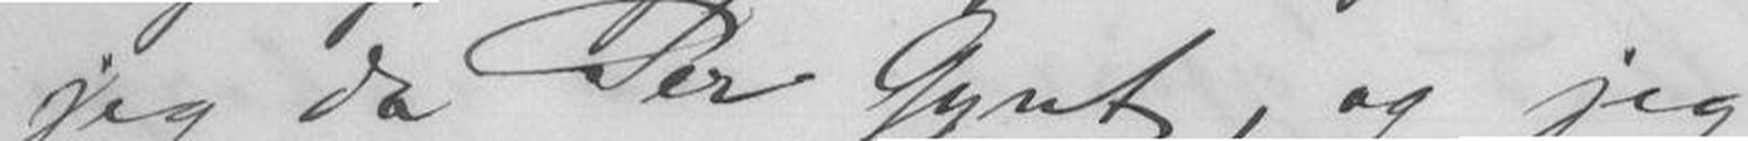jeg da Per Gynt, og jeg
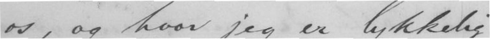os, og hvor jeg er lykkelig
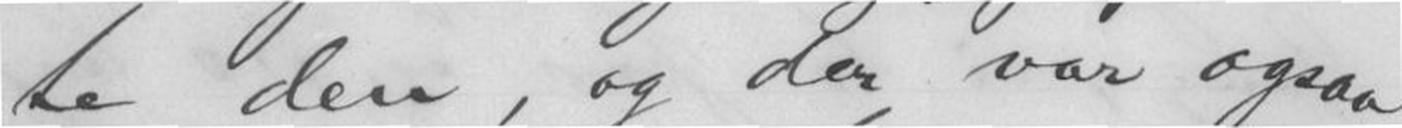te den, og der var ogsaa
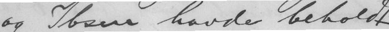og Ibsen havde beholdt
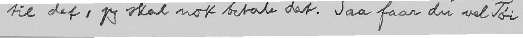til det, jeg skal nok betale det. Saa faar du vel Tøi
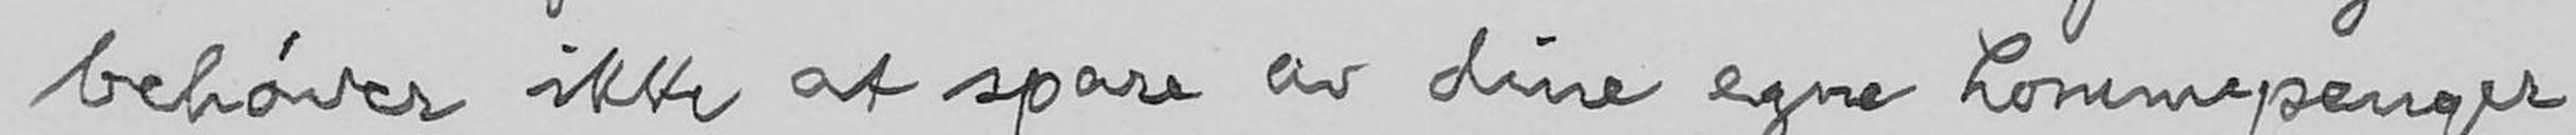behøver ikke at spare av dine egne Lommepenger
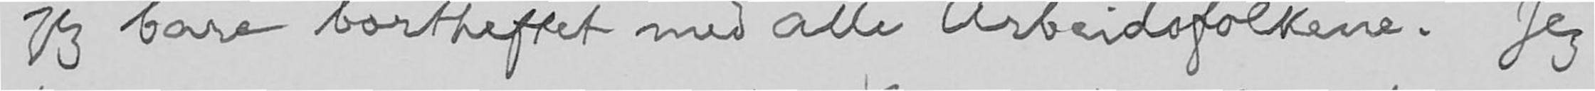jeg bare bortheftet med alle Arbeidsfolkene. Jeg
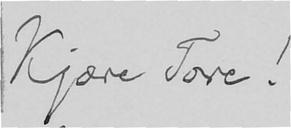Kjære Tore!
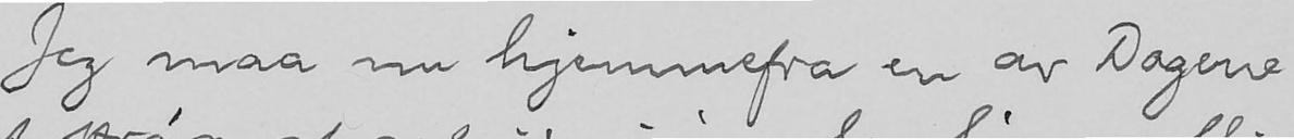Jeg maa nu hjemmefra en av Dagene
